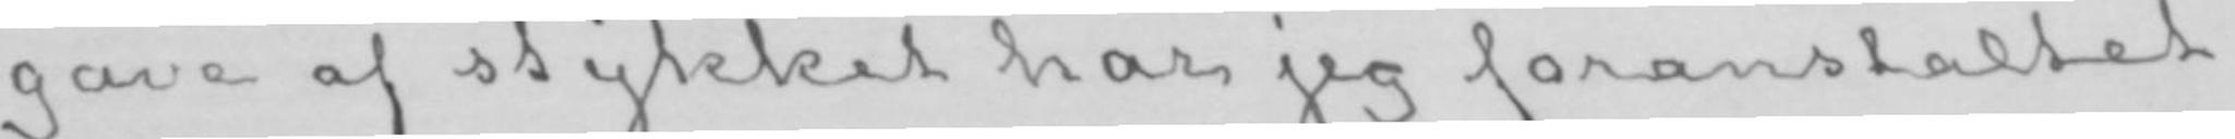gave af stykket har jeg foranstaltet
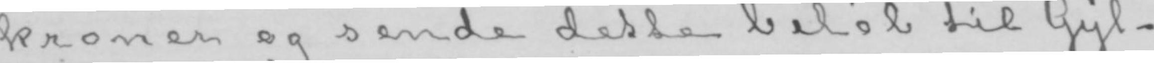kroner og sende dette beløb til Gyl-
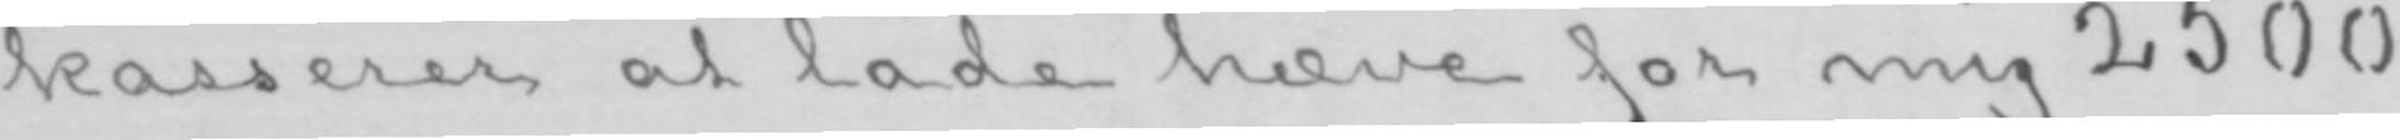kasserer at lade hæve for mig 2500
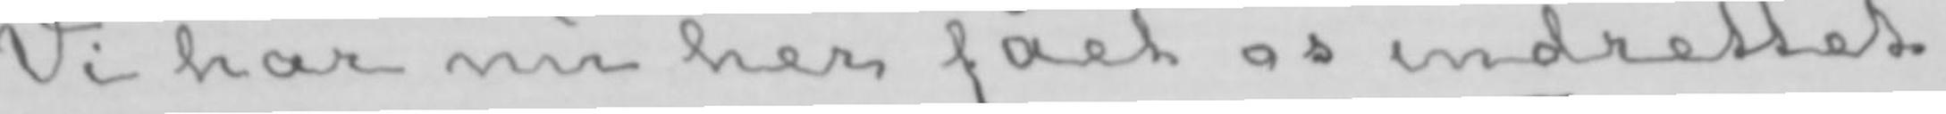Vi har nu her fået os indrettet
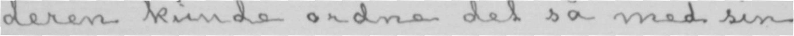deren kunde ordne det så med sin
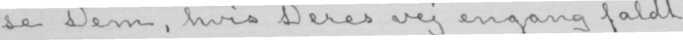se Dem, hvis Deres vej engang faldt
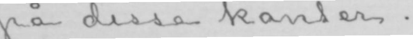på disse kanter.
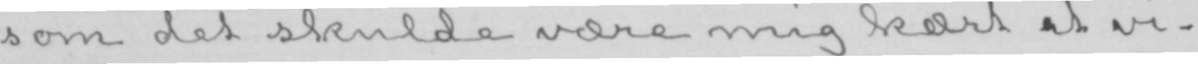som det skulde være mig kært at vi-
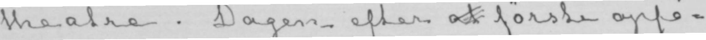theatre. Dagen efter at første opfø-
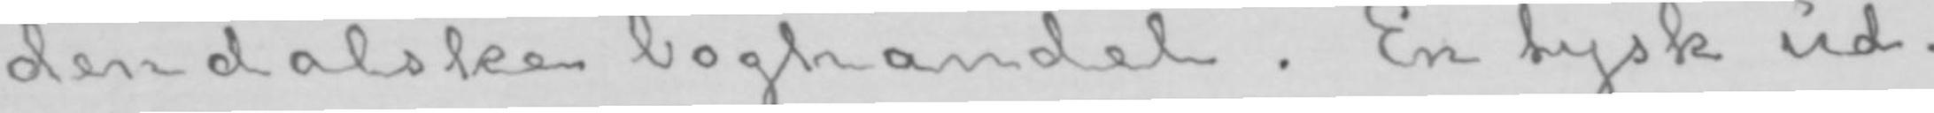dendalske boghandel. En tysk ud-
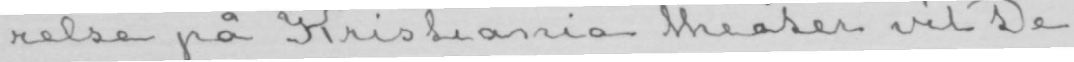relse på Kristiania theater vil De
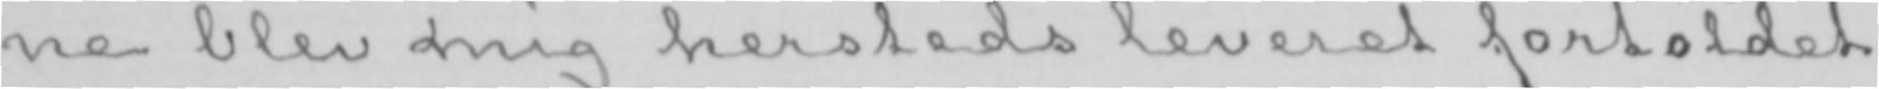ne blev mig hersteds leveret fortoldet
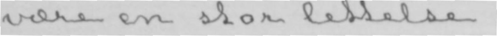være en stor lettelse.
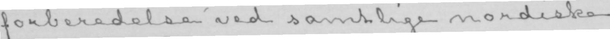forberedelse ved samtlige nordiske
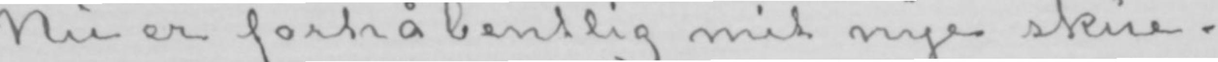Nu er forhåbentlig mit nye skue-
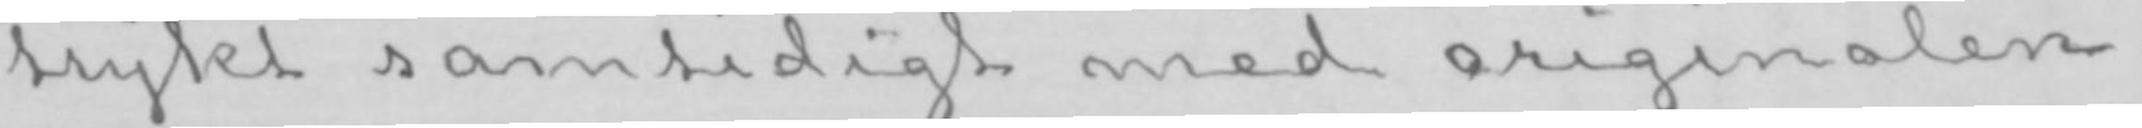trykt samtidigt med originalen.
